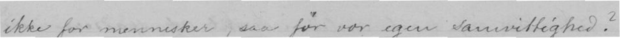ikke for mennesker, saa før vor egen samvittighed?
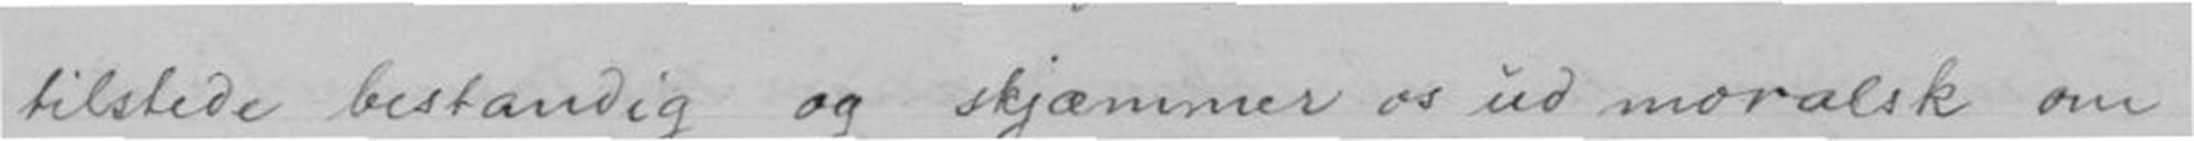tilstede bestandig og skjæmmer os ud moralsk om
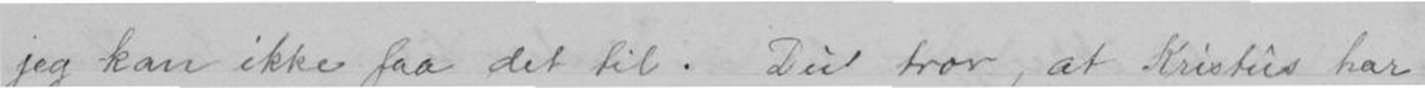jeg kan ikke faa det til. Du tror, at Kristus har
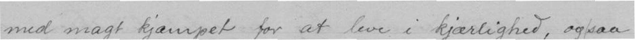med magt kjæmpet for at leve i kjærlighed, og saa
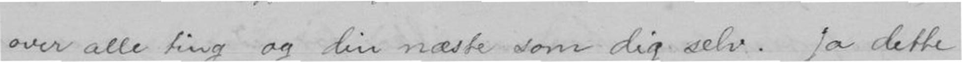over alle ting og den næste som dig selv. Ja dette
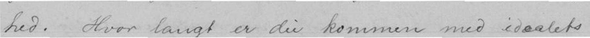hed. Hvor langt er du kommen med idealets
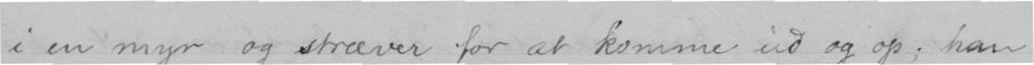i en myr og stræver for at komme ud og op; han
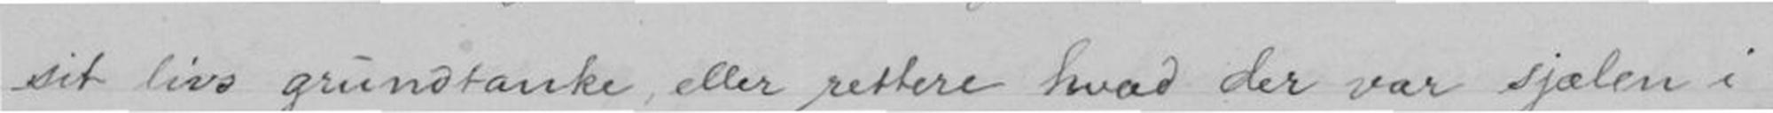sit livs grungtanke, eller rettere hvad der var sjælen i
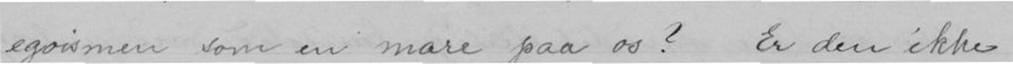egoismen som en mare paa os? Er den ikke
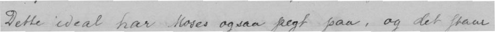Dette ideal har Moses ogsaa pegt paa, og det staar
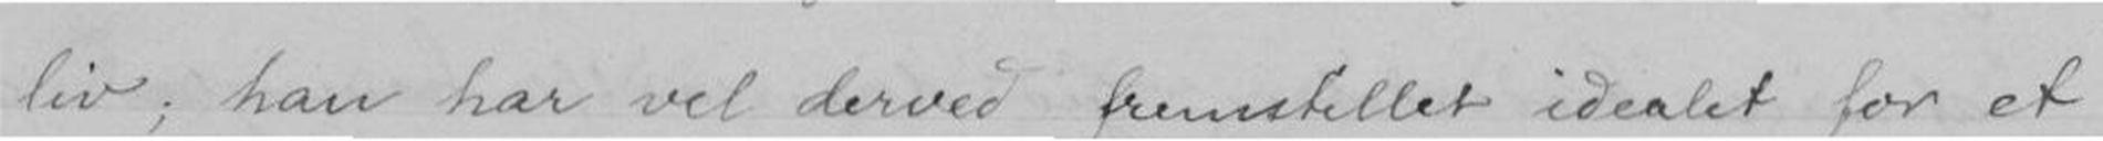liv; han har vel derved frenstillet idealet for et
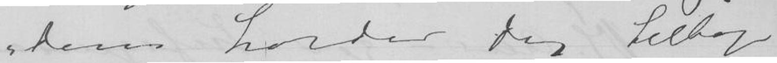dom holder Dig tilbage
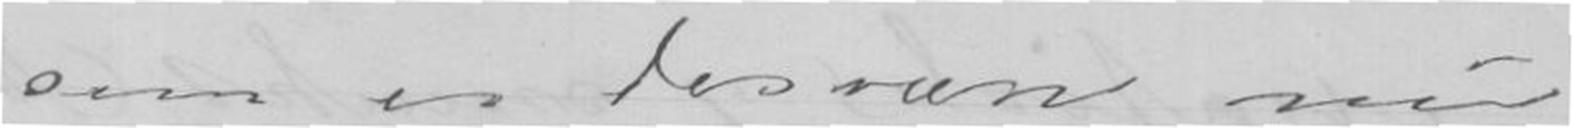som vi desværre nu
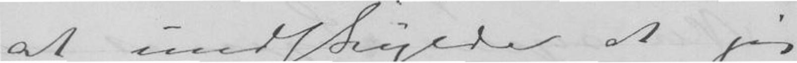at undskylde at jeg
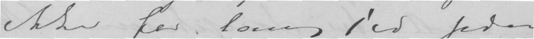ikke for lang Tid siden
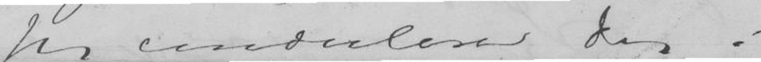Jeg condulderer Dig i
